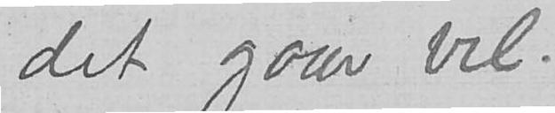Men det gaar vel.
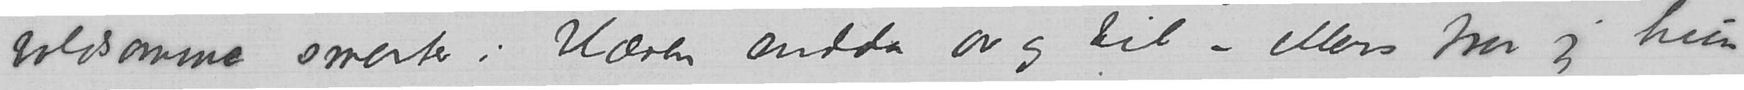voldsomme smerter i blæren endda av og til – ellers tror jeg hun
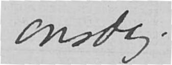onsdag
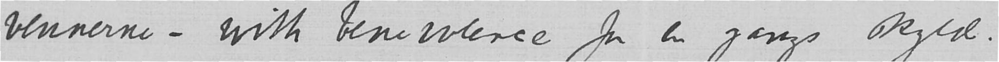vennerne – with benevolence for en gangs skyld.
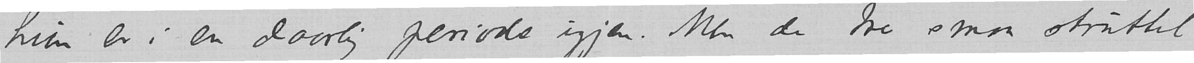hun er i en daarlig periode igjen. Men de tre smaa struttet
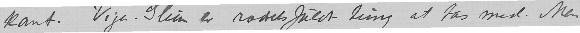kant. Viga-Glum er rædselsfuld tung  at tas med.
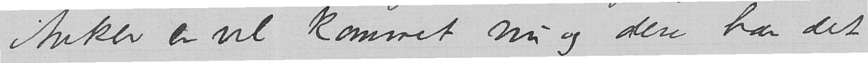Anker er vel kommet nu og dere har det
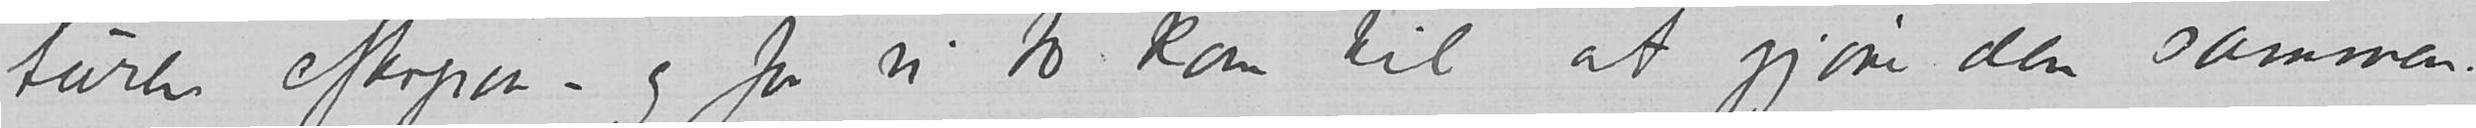turen efterpaa – og for vi to kom til at gjøre den sammen.
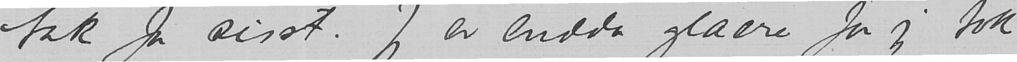tak for sisst. Jeg er endda glaere for jeg tok
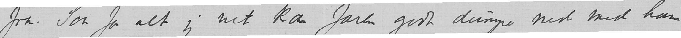fra Saa for alt jeg vet kan faren godt dumpe ned med ham
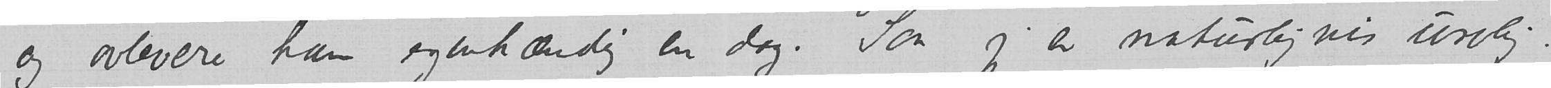og avlevere ham egenhændig en dag. Saa jeg er naturligvis urolig. Ellers kom indmaten
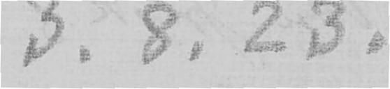3.8.23
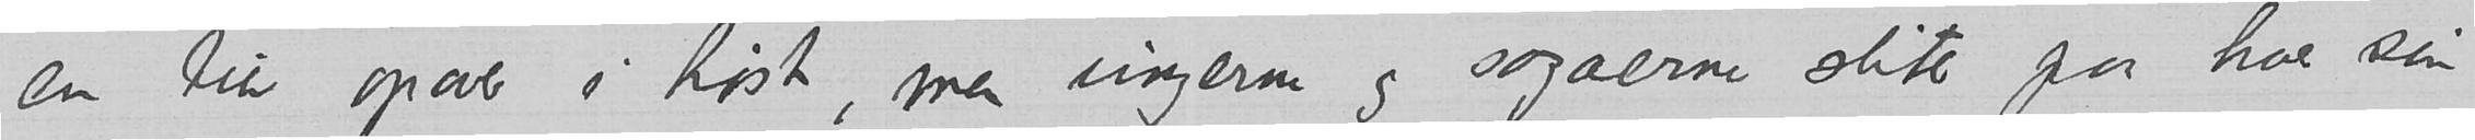en tur opover i høst, men ungerne og sagaerne sliter paa hver sin
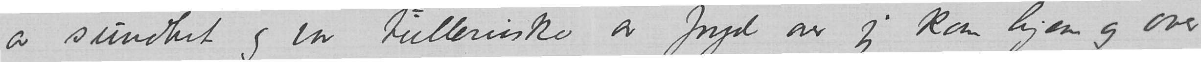av sundhet og var tulleruske av fryd over jeg kom hjem og over
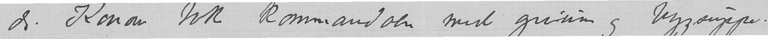dr. Konow tok kommandoen med grinium og bygsuppe .
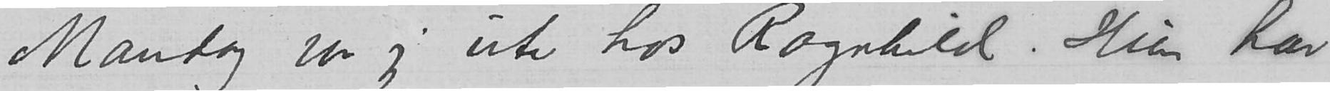Mandag var jeg ute hos Ragnhild. Hun har
